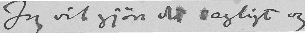Jeg vil gjøre det sagligt og
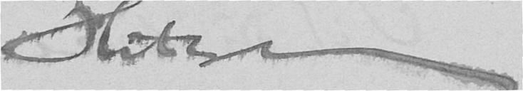Hilsen
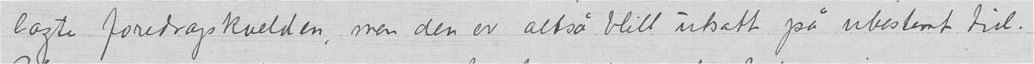lagte foredragskvelden, men den er altså blitt utsatt på ubestemt tid.
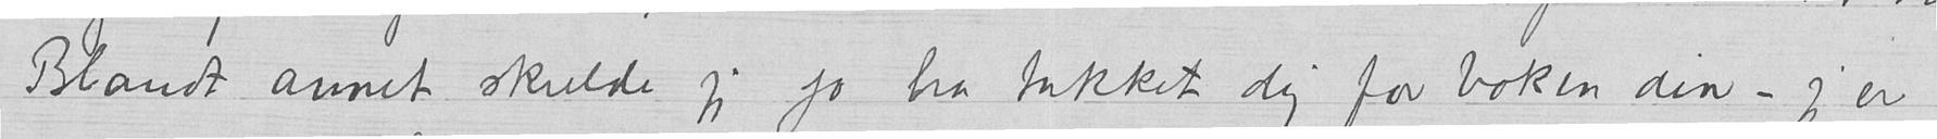Blandt annet skulde jeg jo ha takket dig for boken din – jeg er
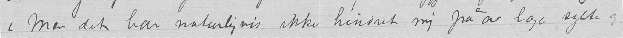(Men det har naturligvis ikke hindret mig fra å lage sylte og
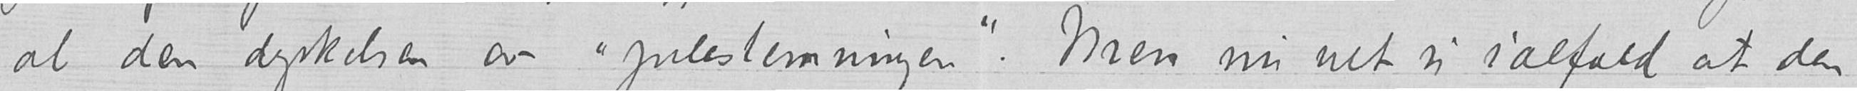al den dyrkelsen av "julestemningen". Men nu vet vi ialfald at den
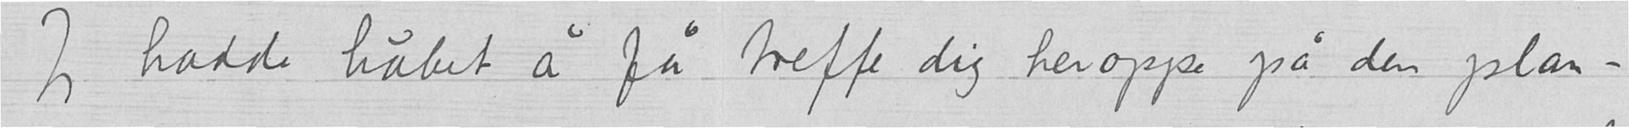Jeg hadde håbet å få treffe dig heroppe på den plan-
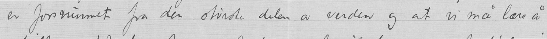er forsvunmet fra den største delen av verden og at vi ma lære å
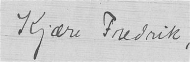Kjære Fredrik,
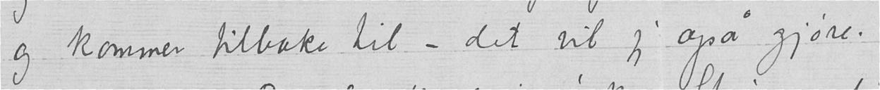og kommer tilbake til – det vil jeg også gjøre.
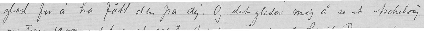glad for å ha fått den fra dig. Og det gleder mig å se at Aschehoug
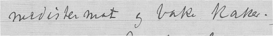medistermat og bake kaker.
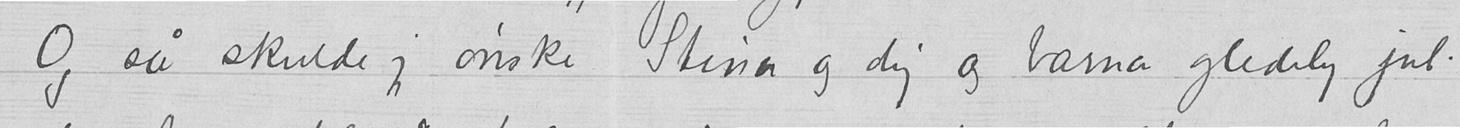Og så skulde jeg ønske Stina og dig og barna gledelig jul.
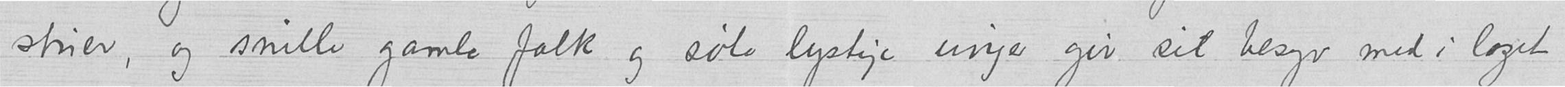stuer, og snille gamle folk og søte lystige unger gir sit besyv med i laget
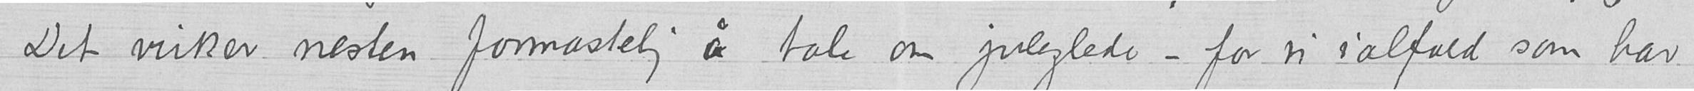Det virker nesten formastelig å tale om juleglede – for vi ialfald som har
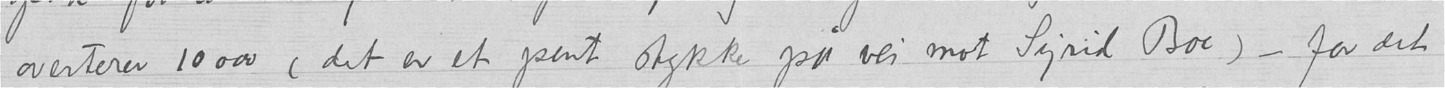averterer 10 000 (det er et pent stykke paa vei mot Sigrid Boe) – for det
